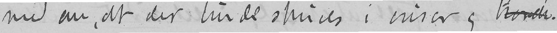med om, at der burde skrives i aviser og karach
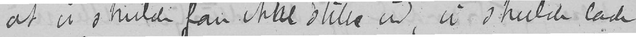at vi skulde fan ikke stille ud, vi skulde lade
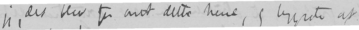jeg, det blev for ondt dette had, og begyndte at
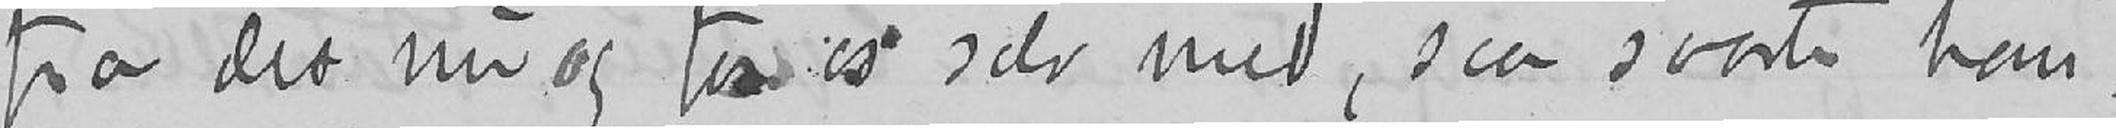fra det nu og for os selv med, saa svarte han,
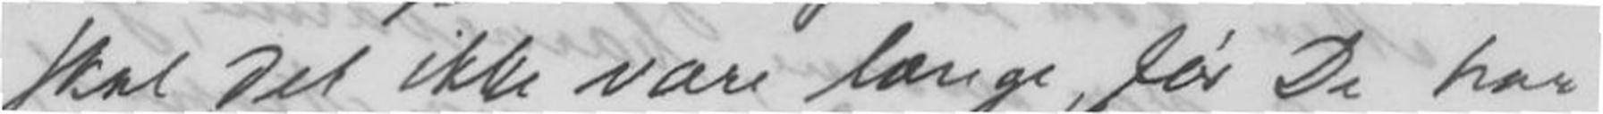skal det ikke vare længe, før De har
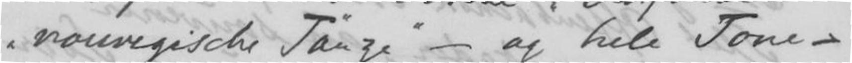nouvegische Tanze - og hele Tone-
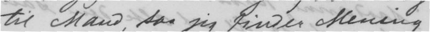til Mand, saa jeg finder Mening
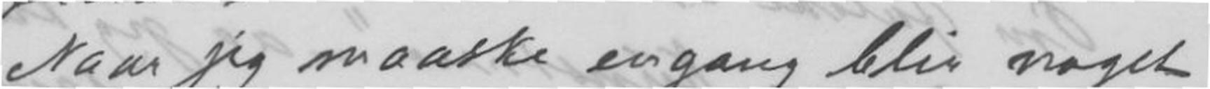Naar jeg maaske engang blir noget
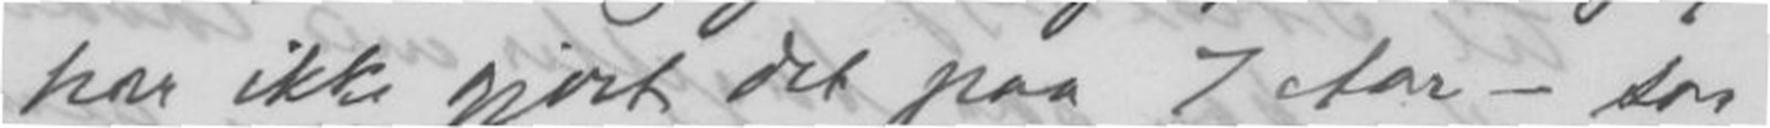har ikke gjort det paa 7 Aar - saa
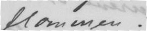flommen!
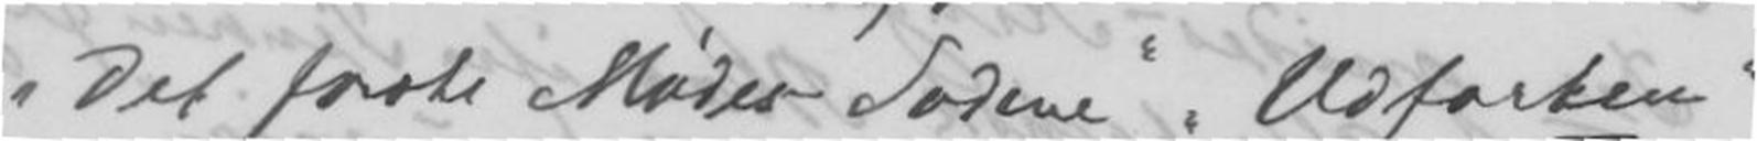Det første Mødes sødme, Udfarten
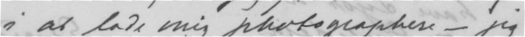i at lade mig photographere - jeg
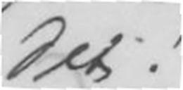det !
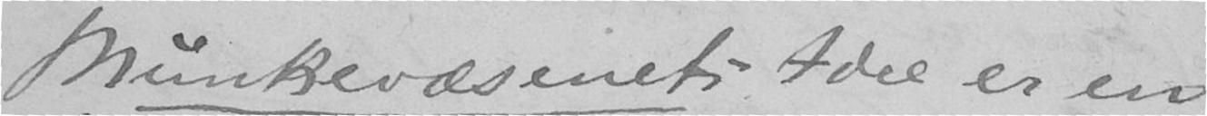Munkevæsenets Idee er en
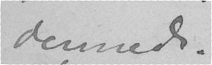dermed.
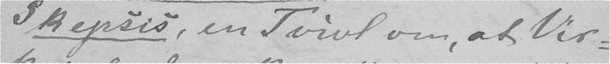Skepsis, en Tvivl om, at Vir-
